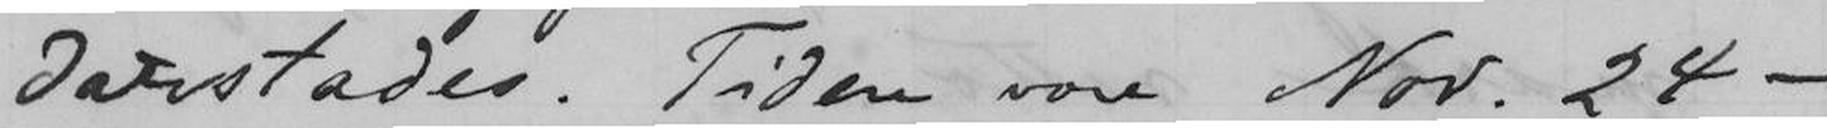Därtades. Tiden vore Nov. 24 -
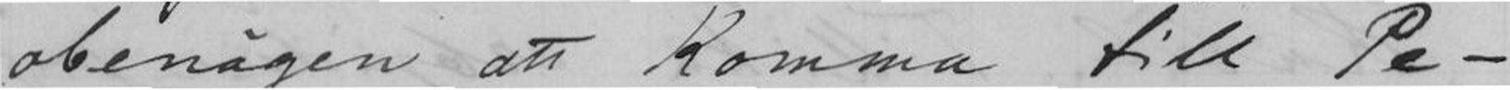obenägen att komma till Pe-
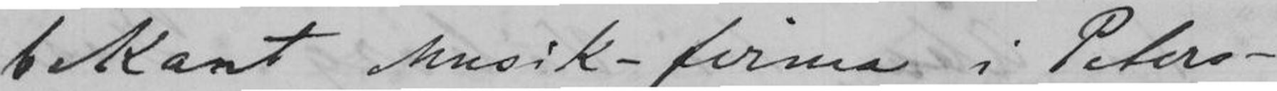bekant Musik-firma i Peters-
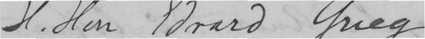H. Herr Edvard Grieg
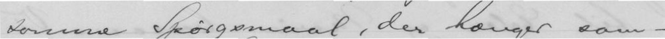somme Spørsmaal, der hænger sam-
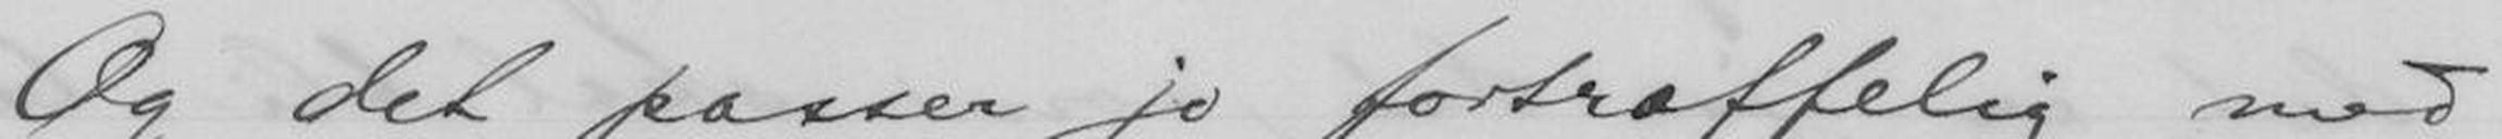Og det passer jo fortræffelig med
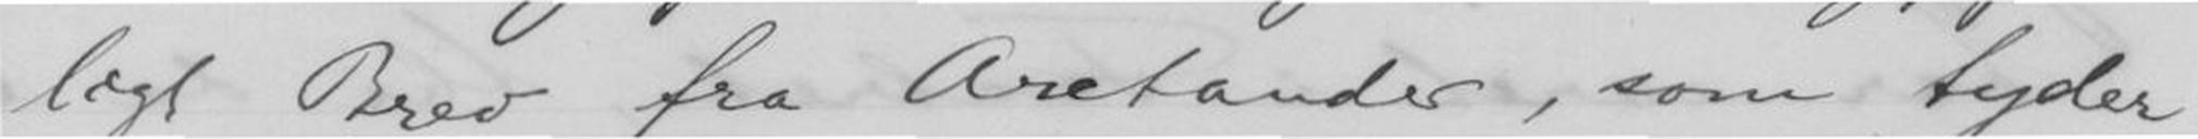ligt Brev fra Arctander, som tyder
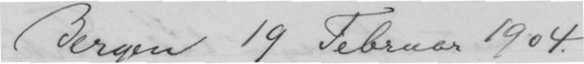Bergen 19 Februar 1904.
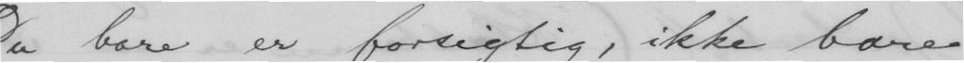Du bare er forsigtig, ikke bare
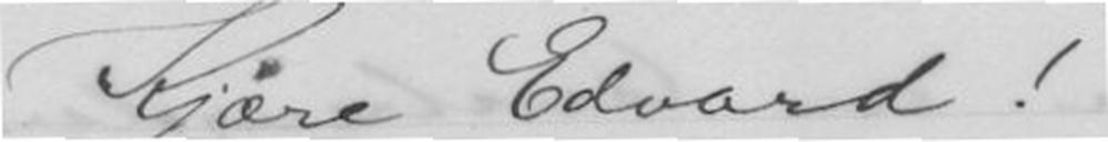Kjære Edvard!
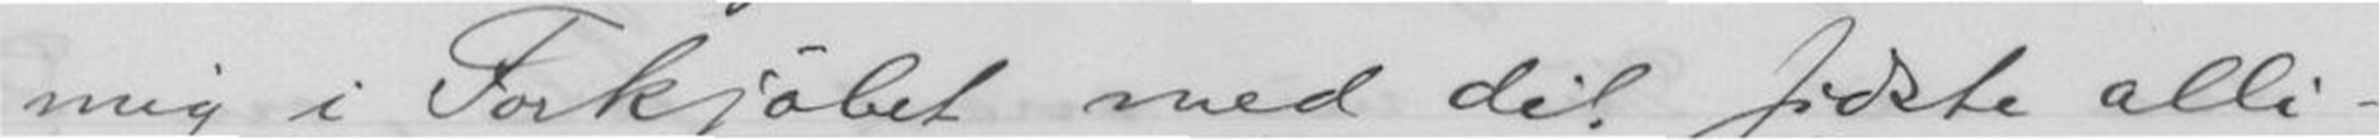mig i Forkjøbet med dit sidste alli-
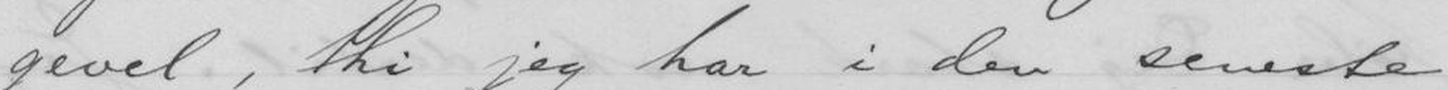gevel, thi jeg har i den seneste
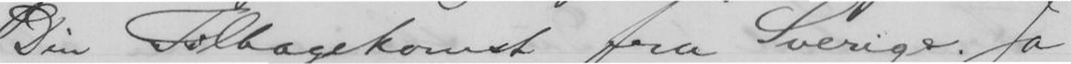Din Tilbagekomst fra Sverige. Ja
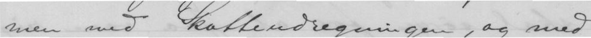men med Skatteudregningen, og med
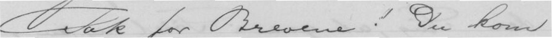Tak for Brevene! Du kom
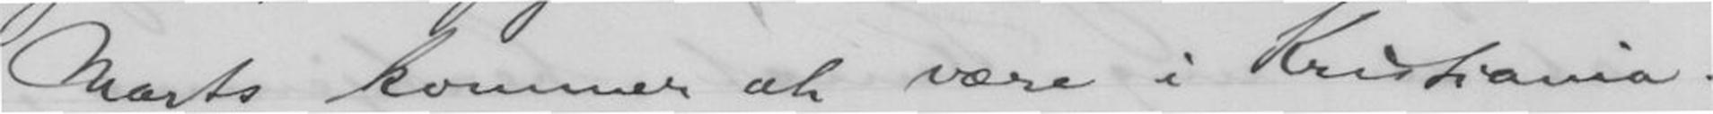Marts kommer at være i Kristiania.
